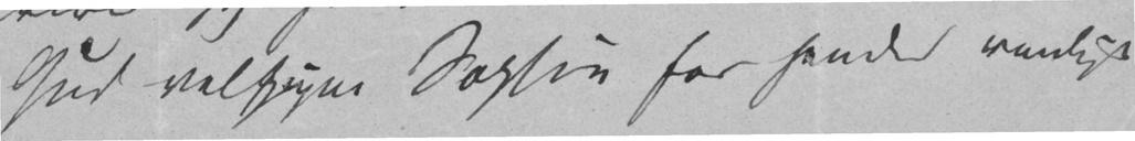Gud velsigne Sophie for hendes venlige
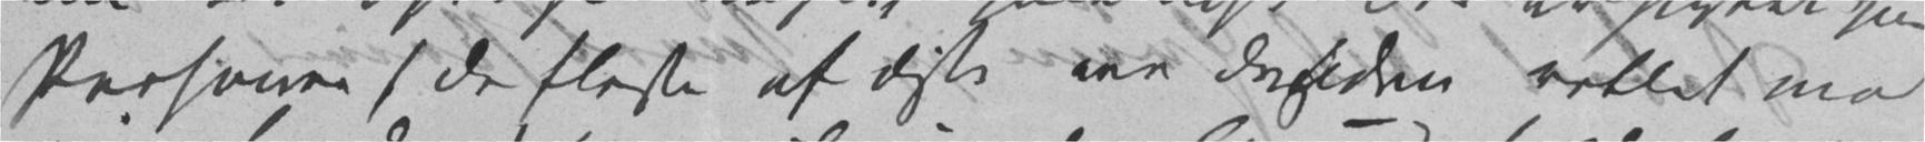Personer (de fleste af disse ere desuden rettet mod
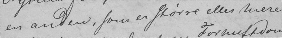en anden, som er større eller mere
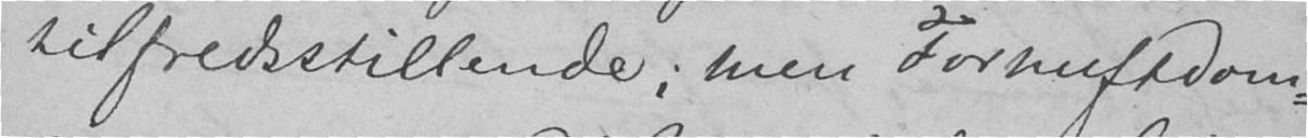tilfredsstillende; men Fornuftdom-
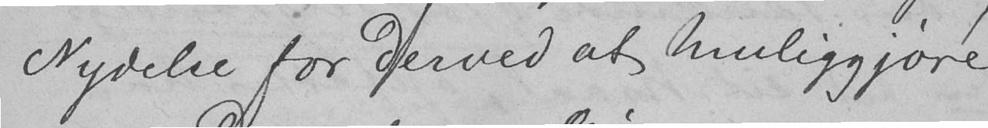Nydelse for derved at muliggjøre
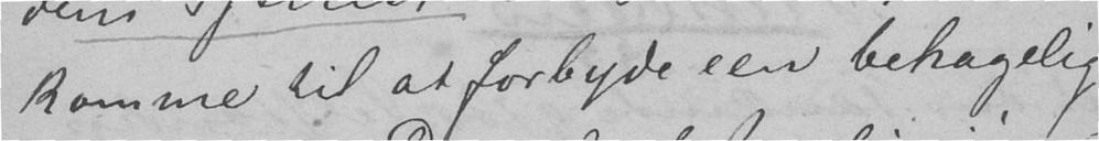komme til at forbyde een behagelig
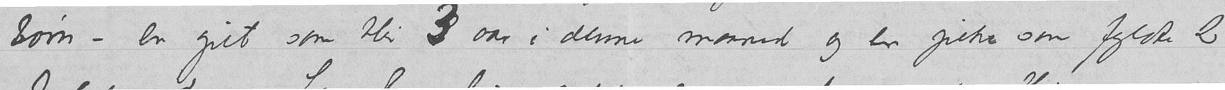børn - en gut som blir 3 aar i denne maaned og en pike som fyldte 2
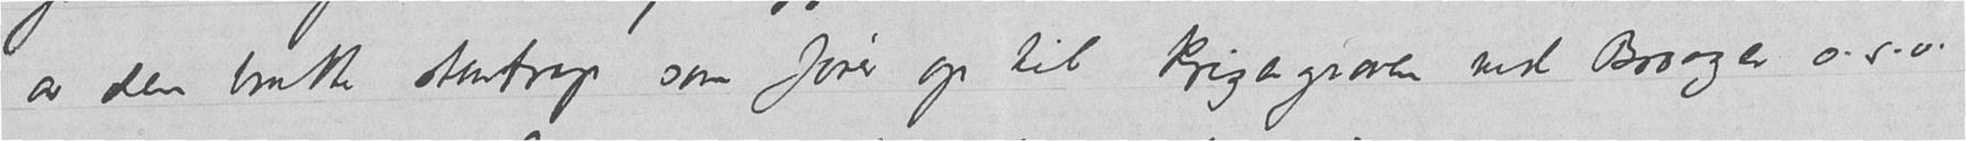av den brutte stentrap som fører op til krigergraven ved Broager o.s.v.
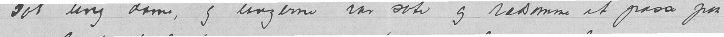søt ung dame, og ungerne var søte og rædsomme at passe paa
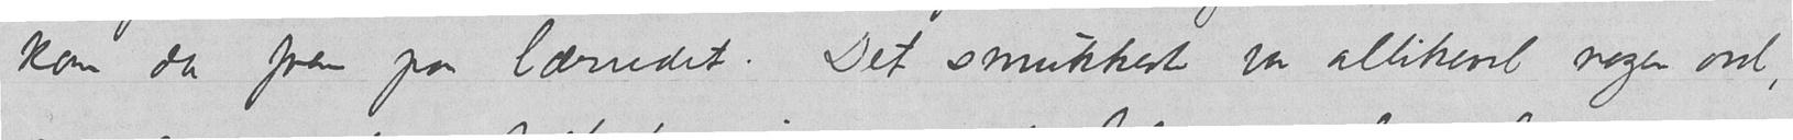kom da frem paa lærredet. Det smukkeste var allikevel nogen ord,
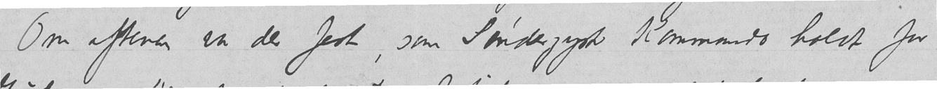Om aftenen var der fest, som Sønderjysk kommando holdt for
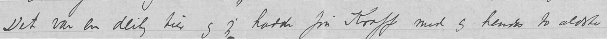Det var en deilig tur og jeg hadde fru Kraft med og hendes to ældste
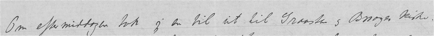Om eftermiddagen tok jeg en bil ut til Graasten og Broager kirke.
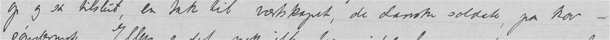op og sa tilslut, en tak til værtskapet, de danske soldater, paa kav
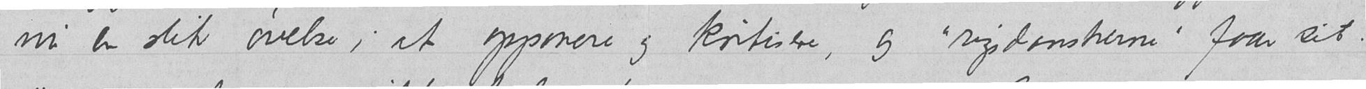nu en slik øvelse i at opponere og kritisere, og "rigsdanskerne" faar sit.
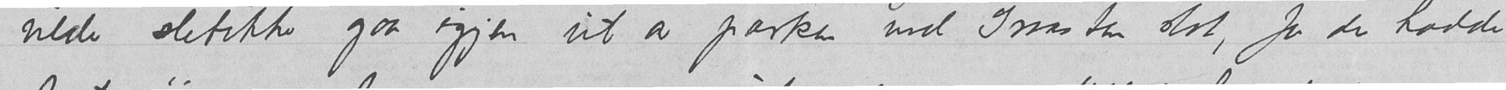vilde sletikke gaa igjen ut av parken ved Graasten slot, for de hadde
